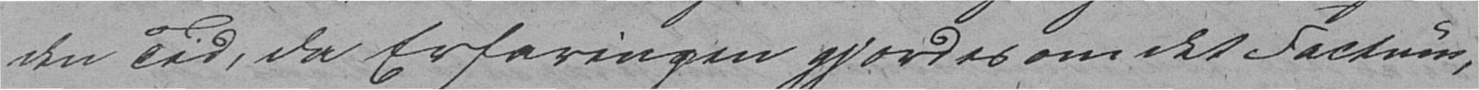den Tid, da Erfaringen gjordes om det Factum,
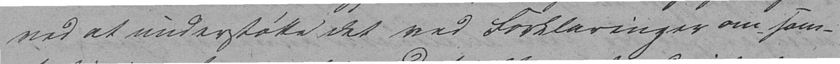ved at understøtte det med Forklaringer om sam-
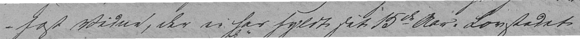fast Vidne, der ei har fyldt sit 15de Aar. Lovstedet
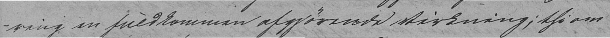ring en fuldkommen afgjørende Virkning; thi om
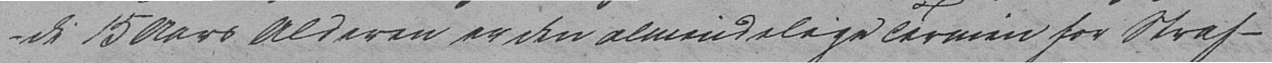di 15 Aars Alderen er den almindelige Termin for Straf-
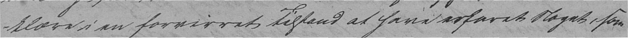klære i en forvirret Tilstand at have erfaret Noget, som
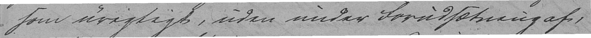som urigtigt, uden under Forudsætning af,
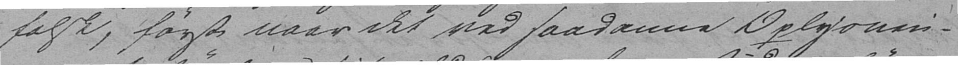falsk, først naar det ved saadanne Oplysnin-
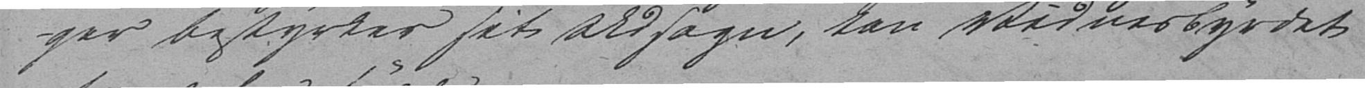ger bestyrker sit Udsagn, kan Vidnesbyrdet
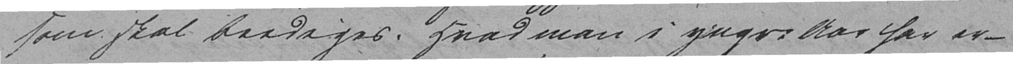som skal beediges. Hvad man i yngre Aar har er-
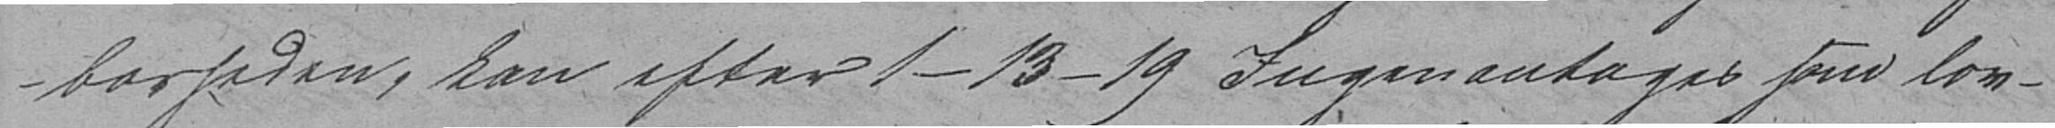barheden, kan efter 1-13-19 Ingen antages som lov-
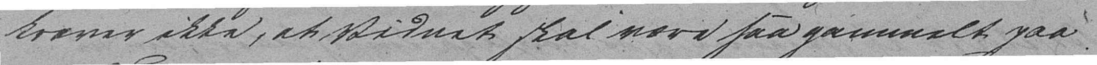kræver ikke, at Vidnet skal være saa gammelt paa
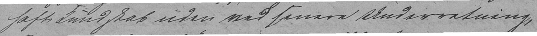haft Kundskab uden ved senere Underretning,
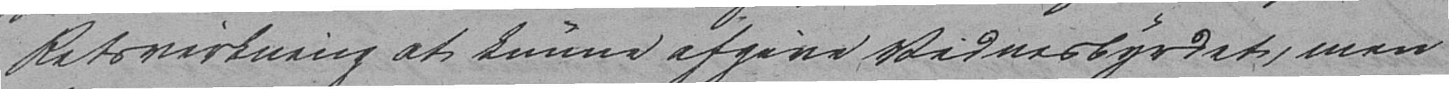Retsvirkning at kunne afgive Vidnesbyrdet, men
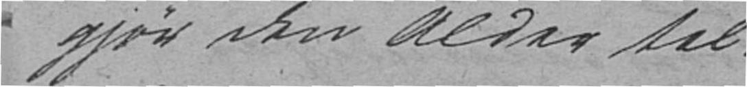gjør den Alder til
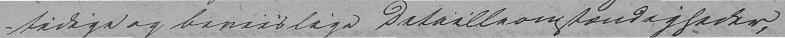tidige og beviislige Detailleomstændigheder,
